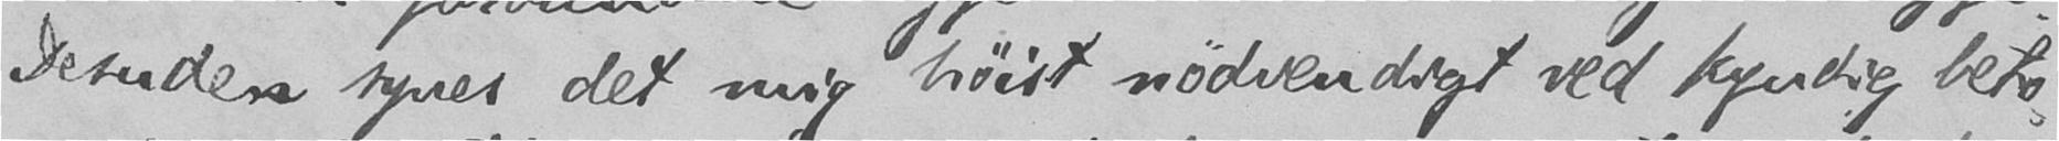Desuden synes det mig høist nødvendigt ved kyndig beto-
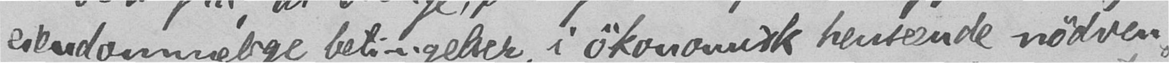eiendommelige betingelser, i økonomisk henseende nødven-
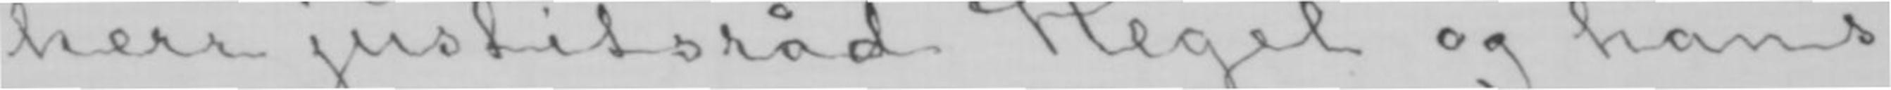herr justitsråd Hegel og hans
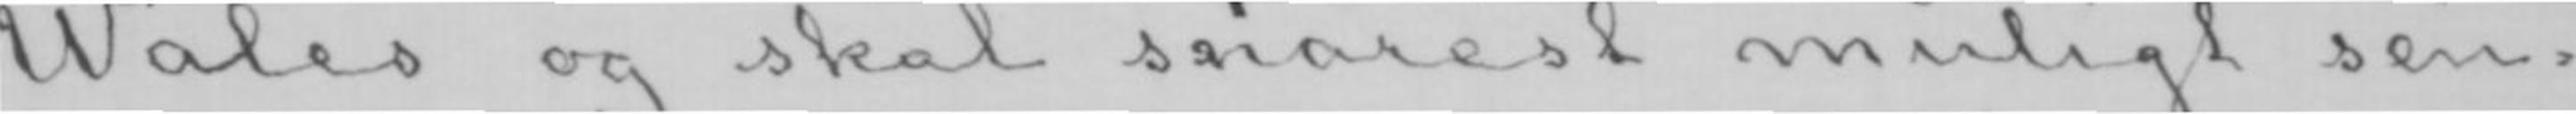Wales og skal snarest muligt sen-
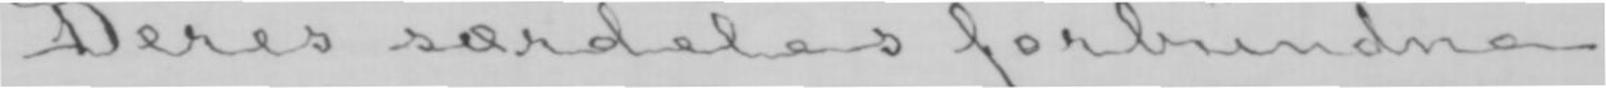Deres særdeles forbundne
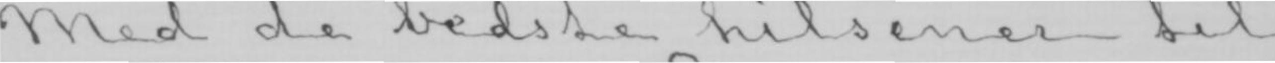Med de bedste hilsener til
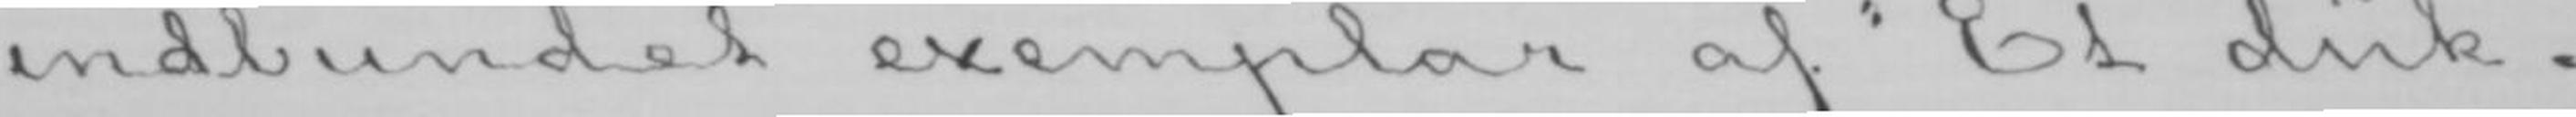indbundet exemplar af «Et duk-
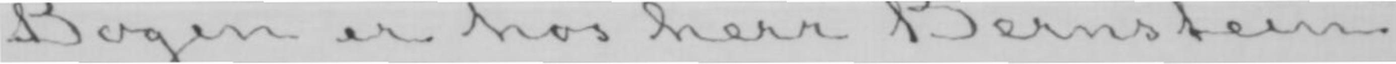Bogen er hos herr Bernstein
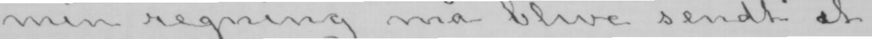min regning må blive sendt et
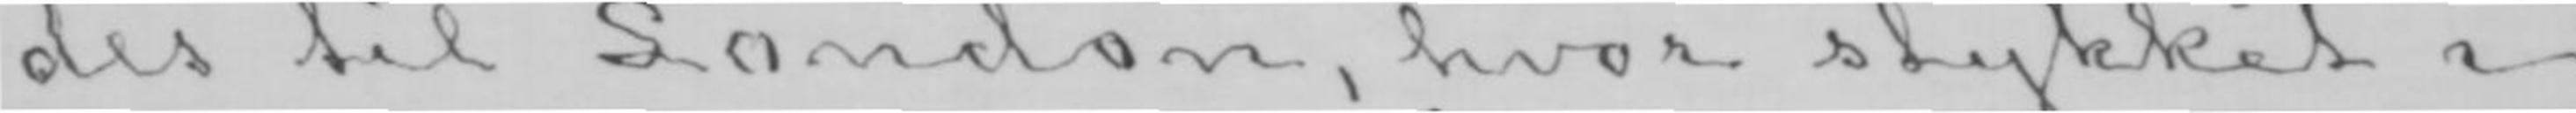des til London, hvor stykket i
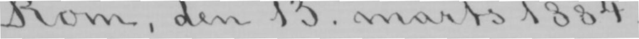Rom, den 13. marts 1884.
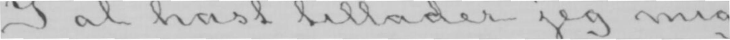I al hast tillader jeg mig
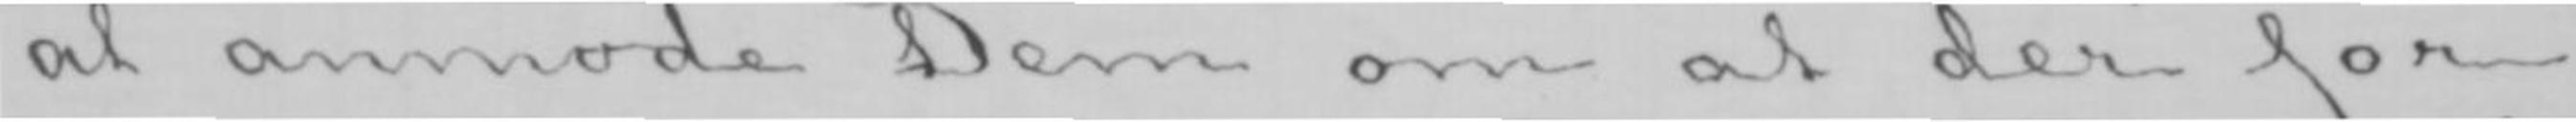at anmode Dem om at der for
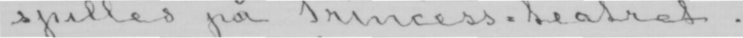spilles på Princess-teatret.
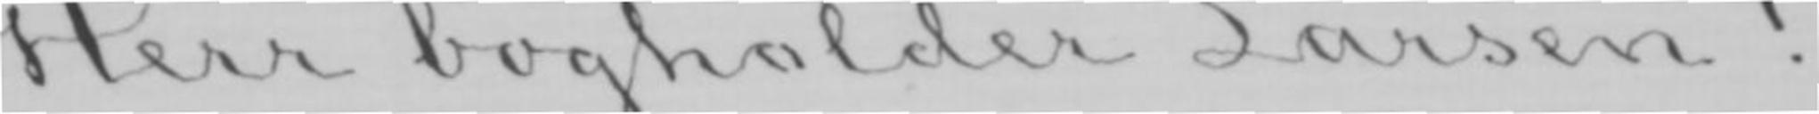Herr bogholder Larsen!
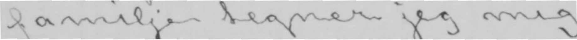familje tegner jeg mig
